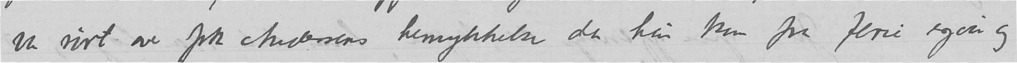var rørt over frk Andersens henrykkelse da hun kom fra ferie igaar og
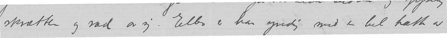skvætten og ræd av sig. Ellers er han yndig med en hel hætte av
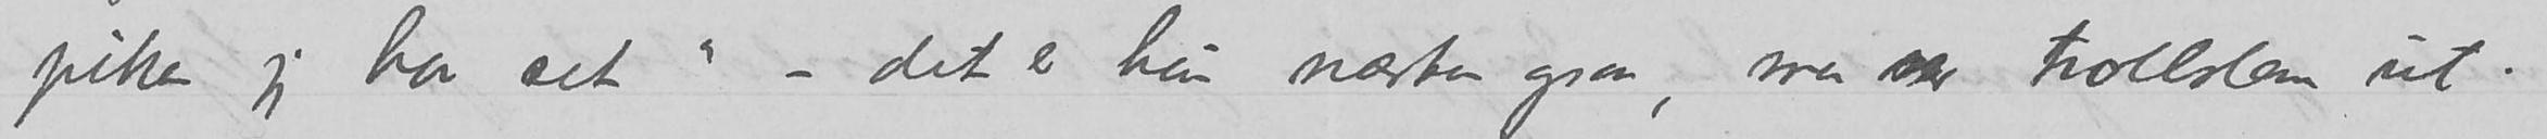piken jeg har set» – det er hun næsten ogsaa, men ser trollslem ut.
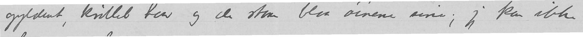gyldent, krøllet hår og de store blaa øinene sine; jeg kan ikke
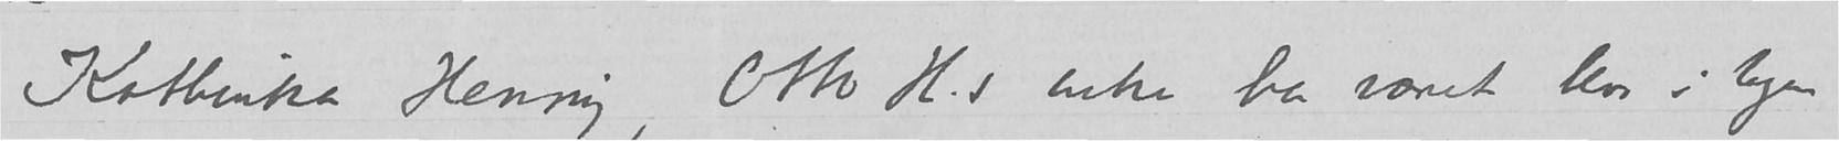Kathinka Hennig, Otto H.s enke har været her i byen
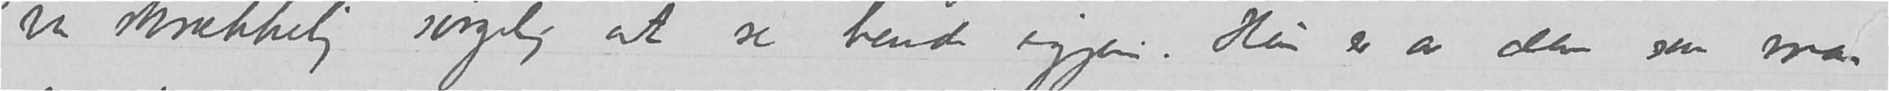var skrækkelig sørgelig at se hende igjen. Hun er av den som man
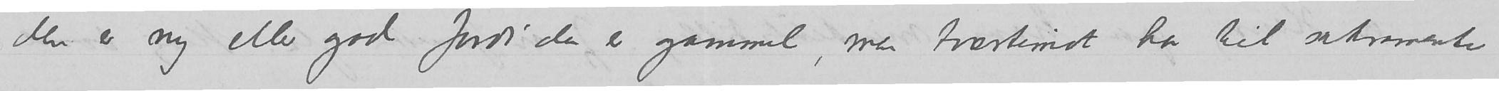den er ny eller god fordi den er gammel, men tværtimot har til sakramenter
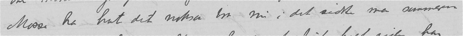Mosse har hat det noksaa bra nu i det sidste men sommeren
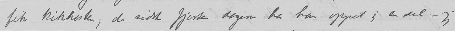fik kikhosten; de sidste fjerten dagene har han oppet sig en del – jeg
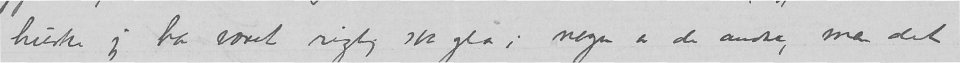huske jeg har været rigtig saa gla i nogen av de andre, men det
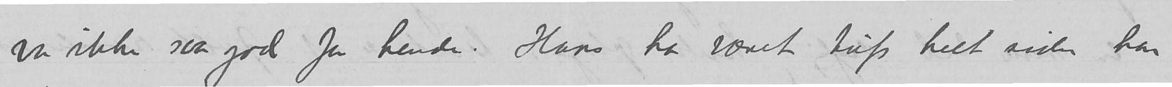var ikke saa god for hende. Hans har været tufs helt siden han
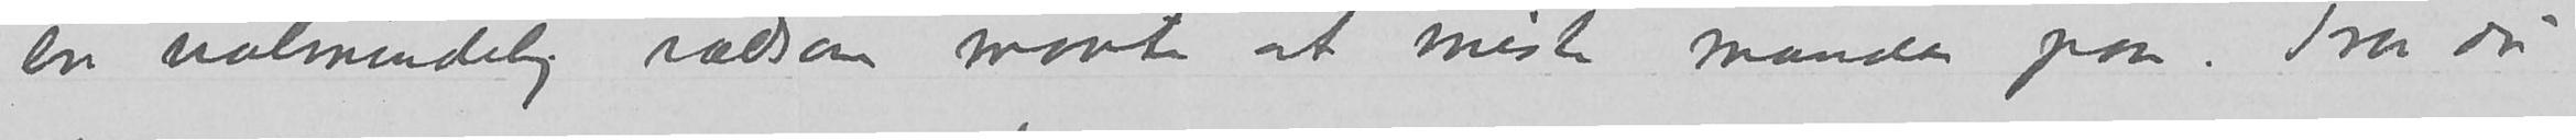en ualmindelig rædsom maate at miste mande paa. Tror du
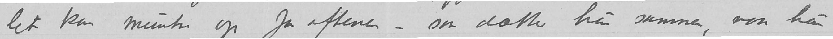let kan muntre op for aftenen – saa dætter hun sammen, naar hun
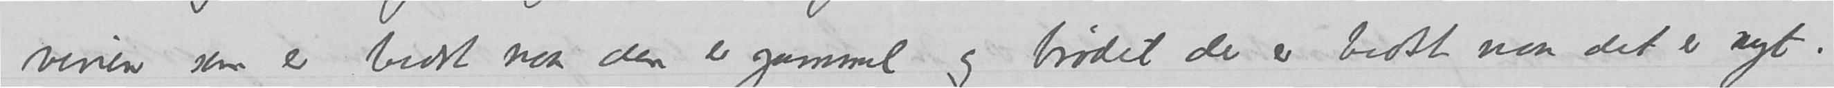vinen som er bedst naar den er gammel og brødet der er bedst naar det er nyt.
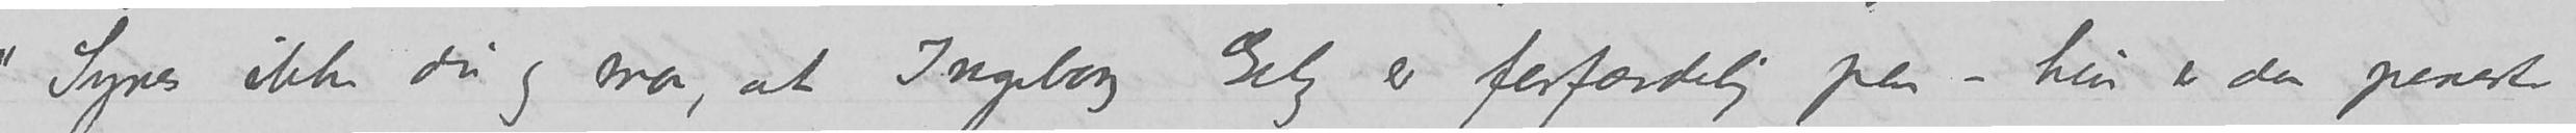«Synes ikke du og mor at, Ingeborg Getz er forfærdelig pen – hun er den peneste
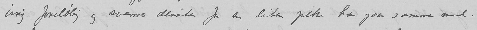ivrig foreløbig og sværmer desuten for en liten pike han gaar sammen med.
